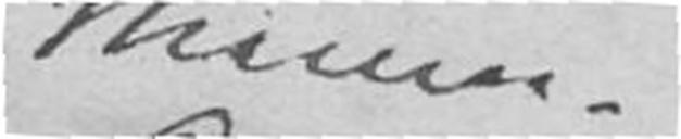Menne-
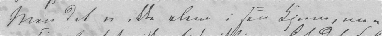Men det er ikke alene i sin Kjerne, men
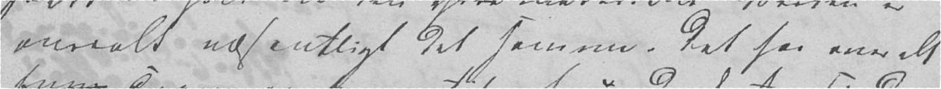overalt væsentligt det samme. Det har overalt
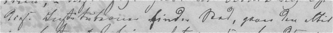disse Institutioner finder Sted, gaaer den altid
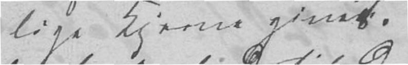lige Kjerne givet.
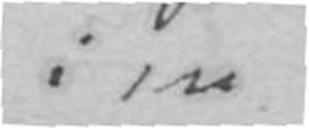i en
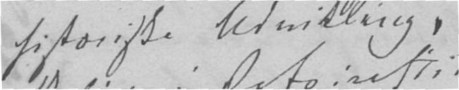historiske Udvikling,
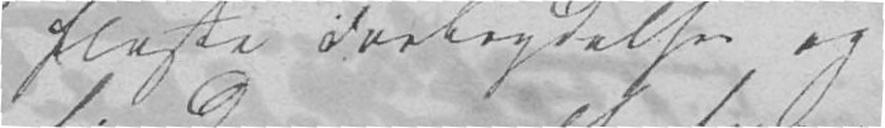fleste Forbrydelser og
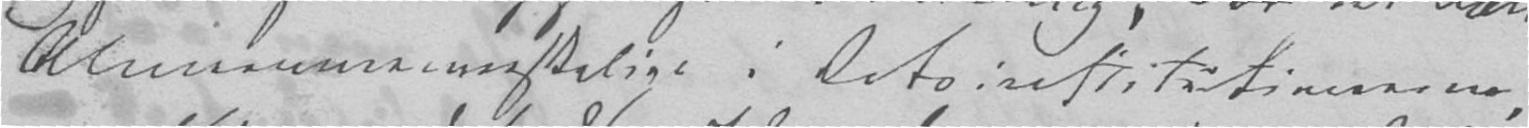Almeenmenneskelige i Retsinstitutionerne,
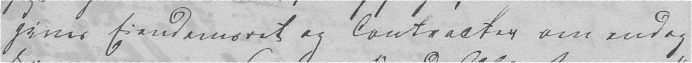gives Eiendomsret og Contracter om endog
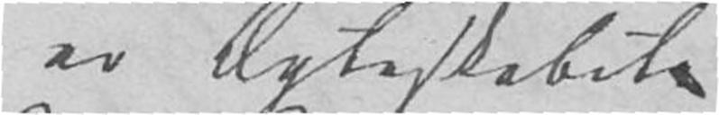er Ægteskabet
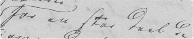for en stor Deel de
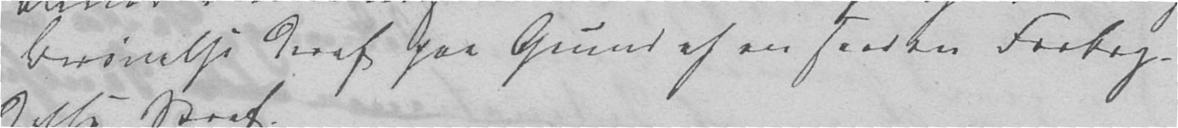Berøvelse deraf paa Grund af en saadan Forbry-
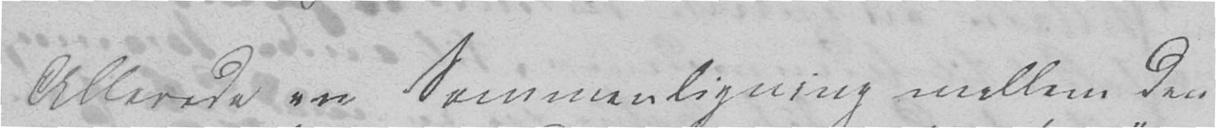Allerede en Sammenligning mellem den
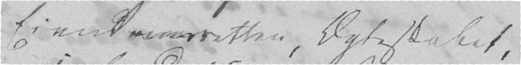Eiendomsretten, Ægteskabet,
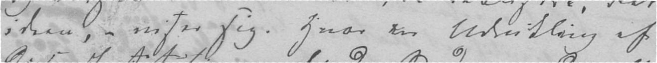ideen, viser sig. Hvor en Udvikling af
